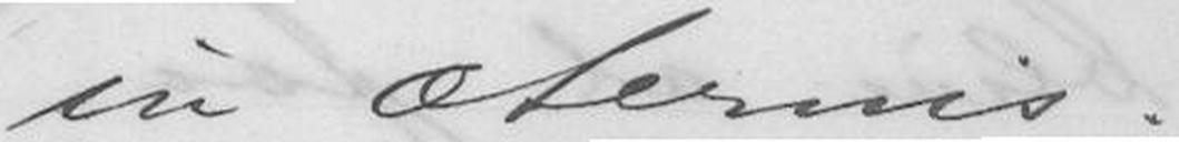in æternis!
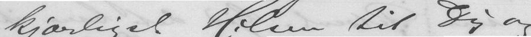kjærligst Hilsen til Dig og
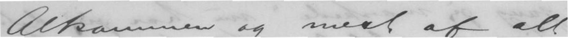Altsammen og mest af alt
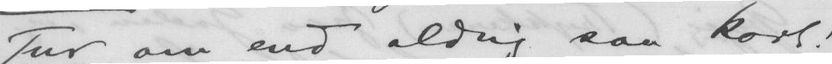Tur om end aldrig saa kort!
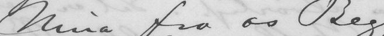Nina fra os Begge!
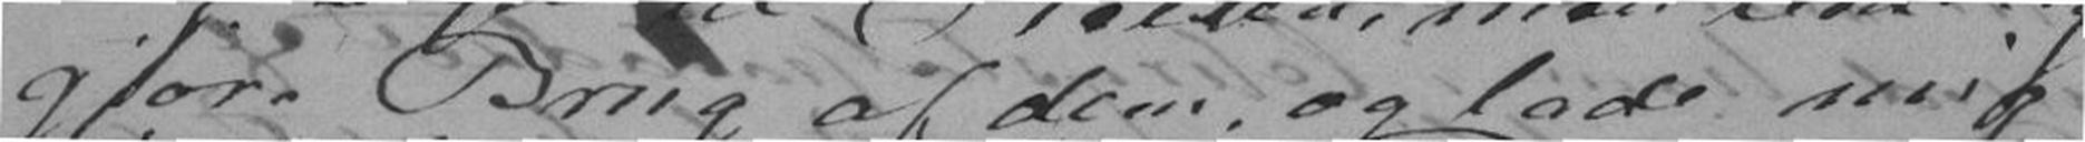gjøre Brug af dem, og lade mig
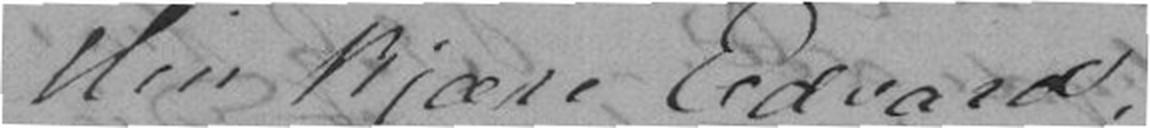Min kjære Edvard!
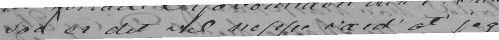saa er det vel neppe værd at jeg
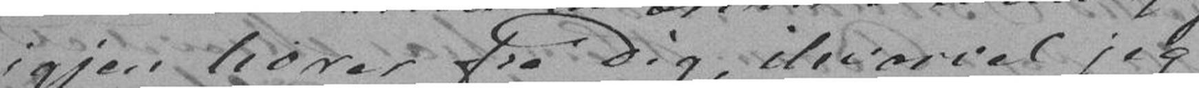igjen hører fra Dig, ihvorvel jeg
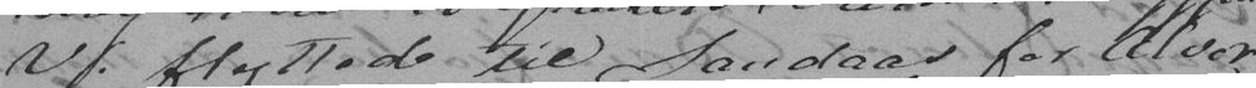Vi flyttede til Landaas for Alvor
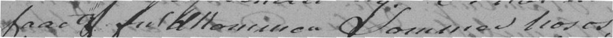faaet fuldkommen Sommer hos os,
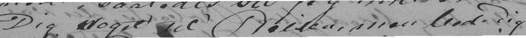Dig noget til Reisen, men bede Dig
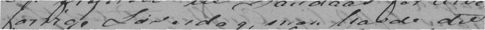forige Løverdag, men havde det
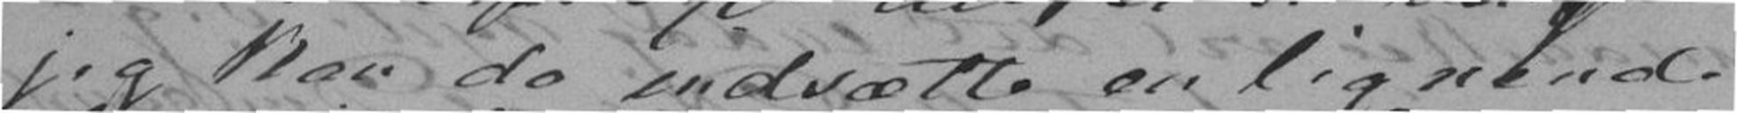jeg kan da indsætte en lignende
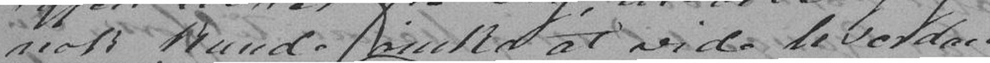nok kunde ønske at vide hvordan
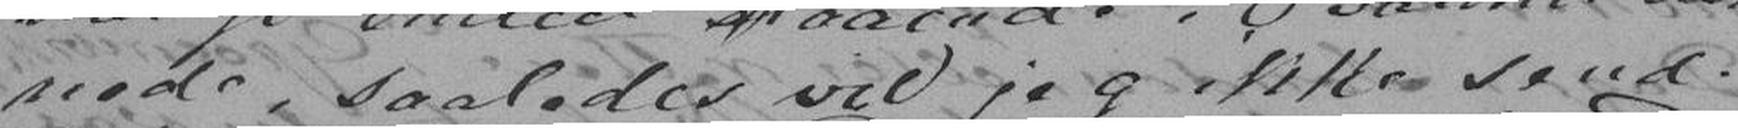nede, saaledes vil jeg ikke sende
